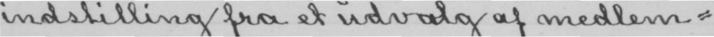indstilling fra et udvalg af medlem-
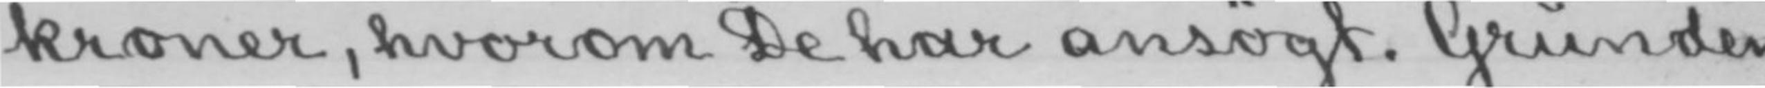kroner, hvorom De har ansøgt. Grunden
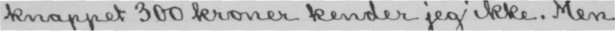knappet 300 kroner kender jeg ikke. Men
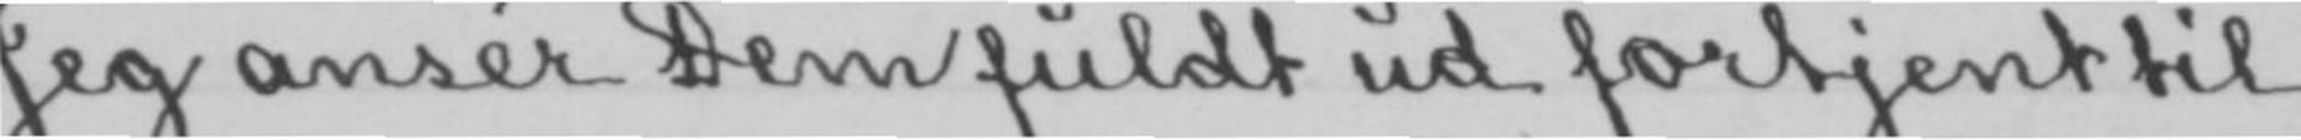Jeg ansér Dem fuldt ud fortjent til
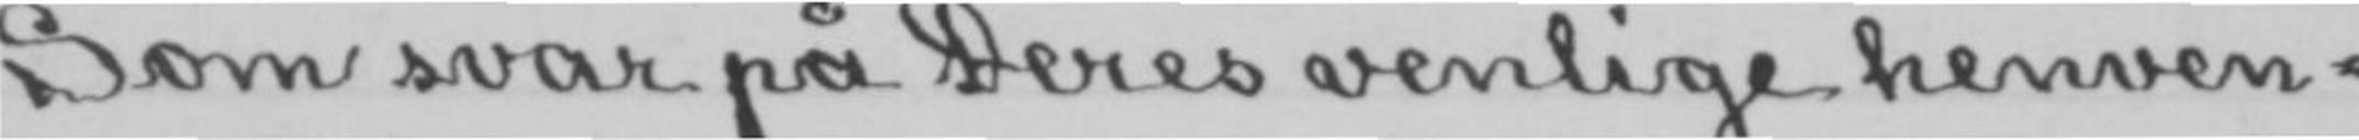Som svar på Deres venlige henven-
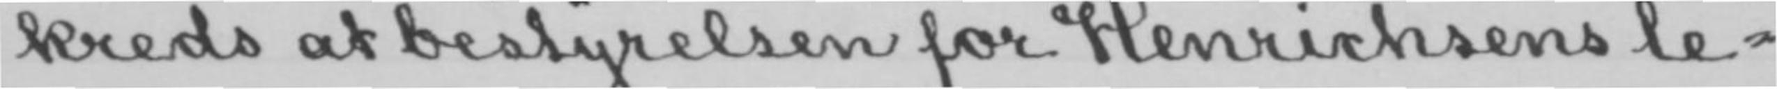kreds at bestyrelsen for Henrichsens le-
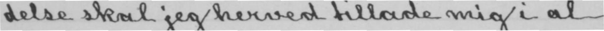delse skal jeg herved tillade mig i al
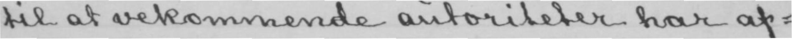til at vekommende autoriteter har af-
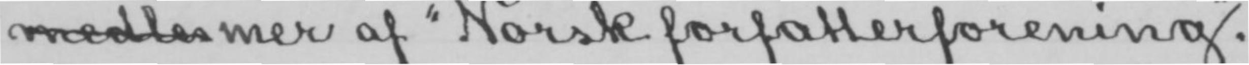medlemer af «Norsk forfatterforening».
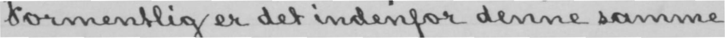Formentlig er det indenfor denne samme
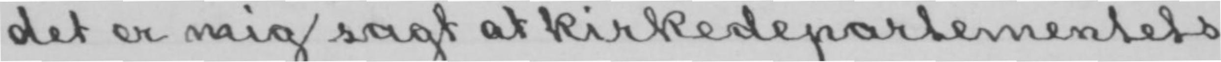det er mig sagt at kirkedepartementets
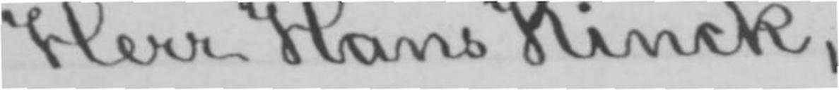Herr Hans Kinck,
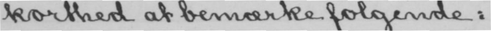korthed at bemærke følgende:
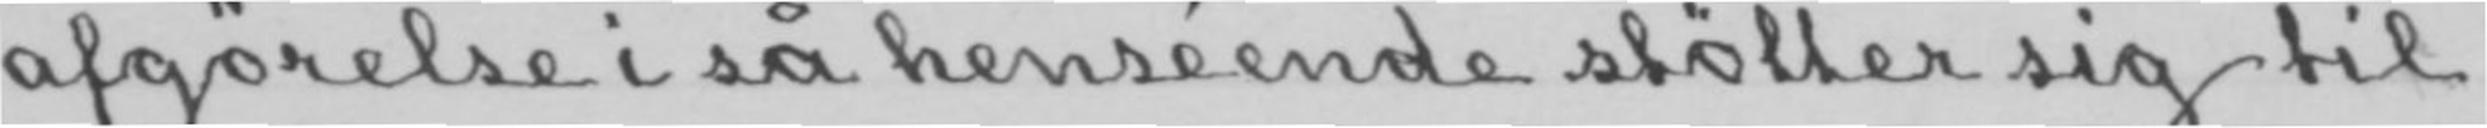afgørelse i så henséende støtter sig til
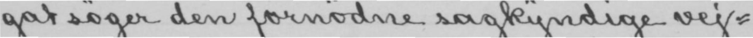gat søger den fornødne sagkyndige vej-
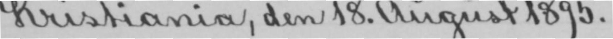Kristiania, den 18. August 1895.
